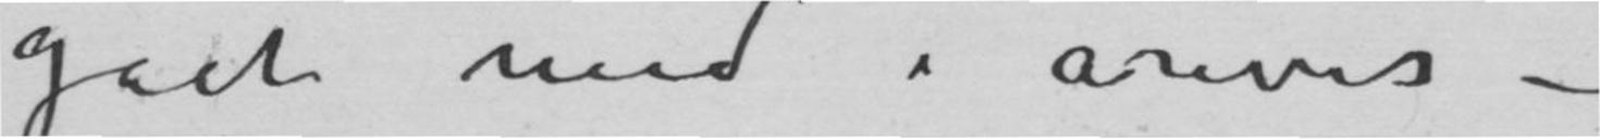gået med i årevis –
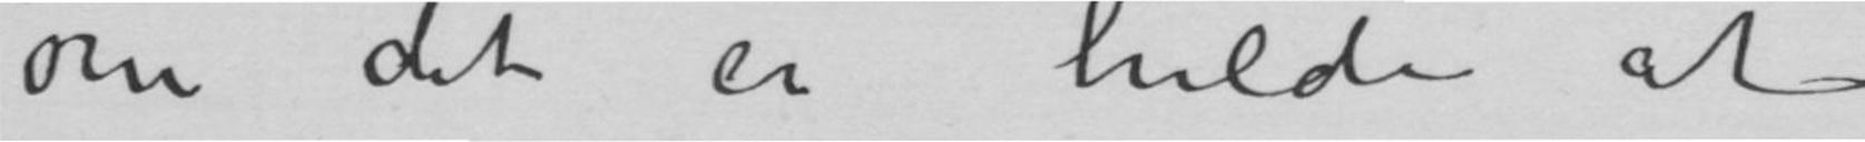om det er heldi at
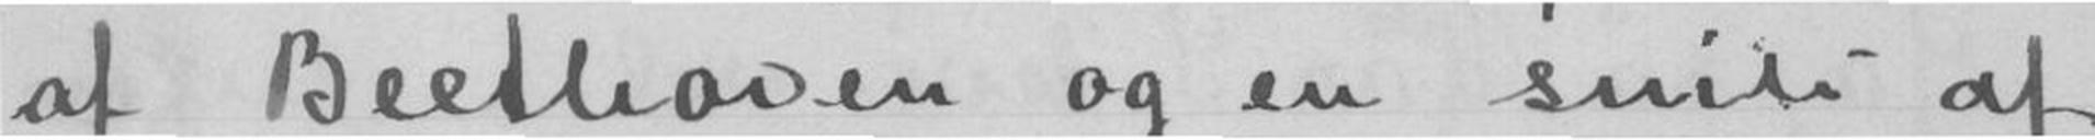af Beethoven og en suite af
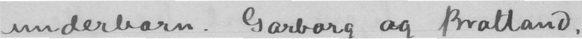underbarn. Garborg og Bratland,
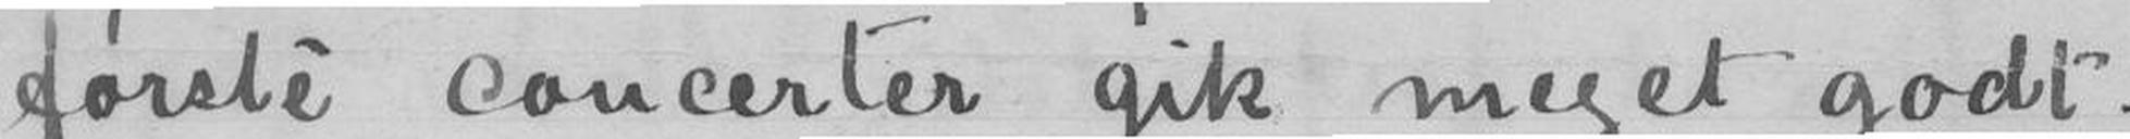første concerter gik meget godt.
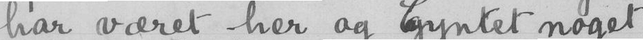har været her og Gyntet noget
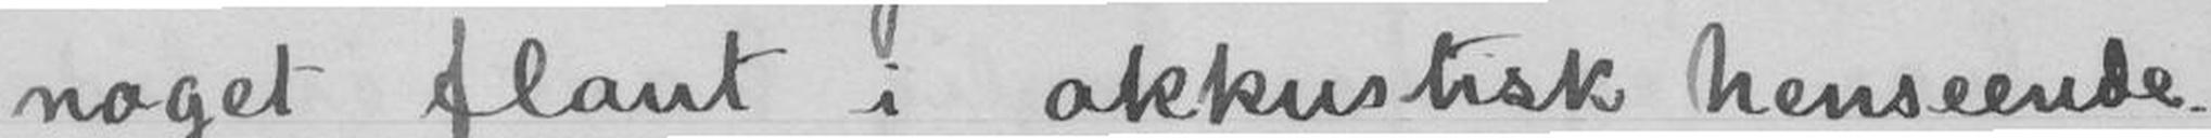noget flaut i akkustisk henseende.
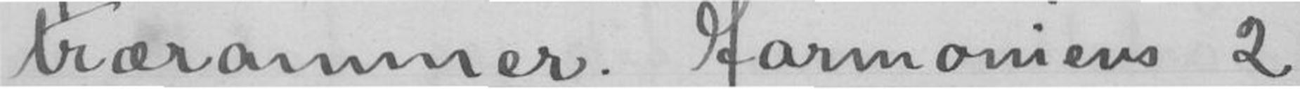trærammer. Harmoniens 2
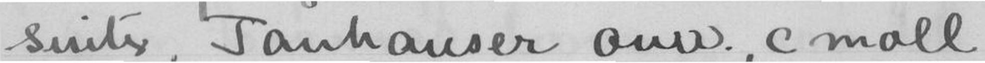suite, Tanhauser ouv., c moll
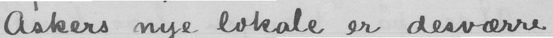Askers nye lokale er desværre
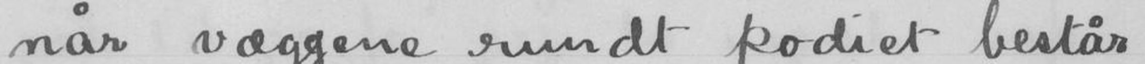når væggene rundt podiet består
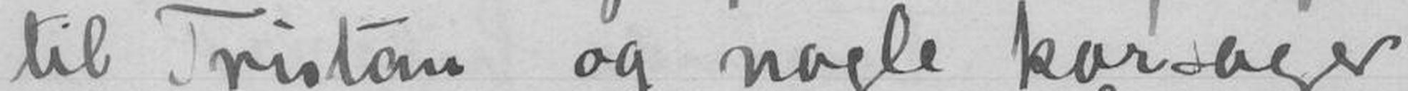til Tristan og nogle korsager
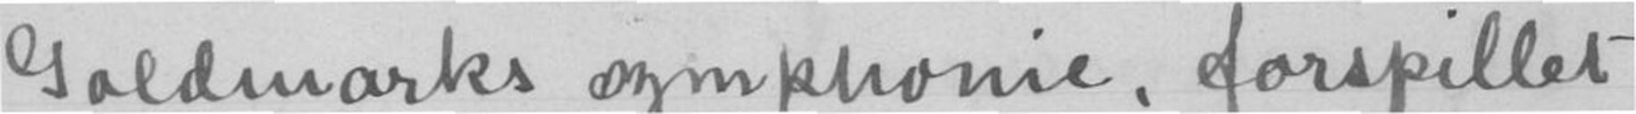Goldmarks symphonie, forspillet
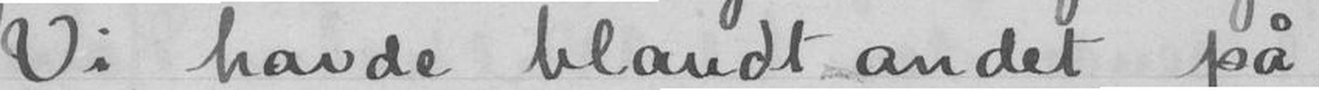Vi havde blandt andet på
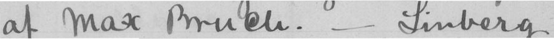af Max Bruck. - Linberg
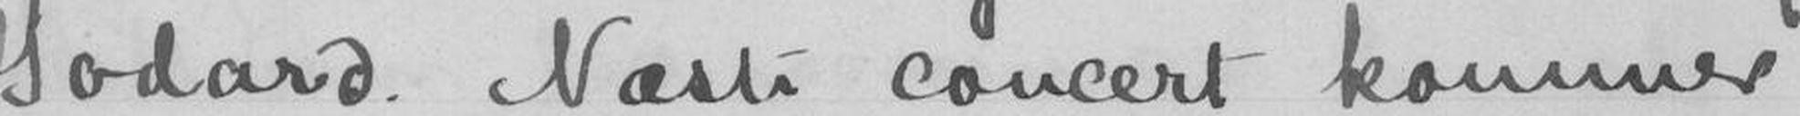Godard. Næste concert kommer
